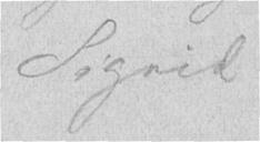Sigrid
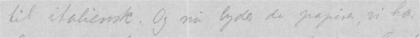til italiensk. Og nu lyder de papirer, vi har
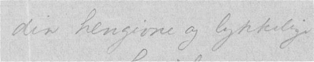din hengivne og lykkelige
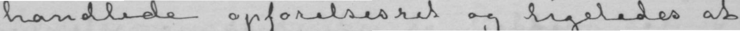handlede opførelsesret og ligeledes at
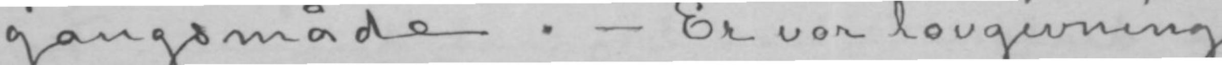gangsmåde. - Er vor lovgivning
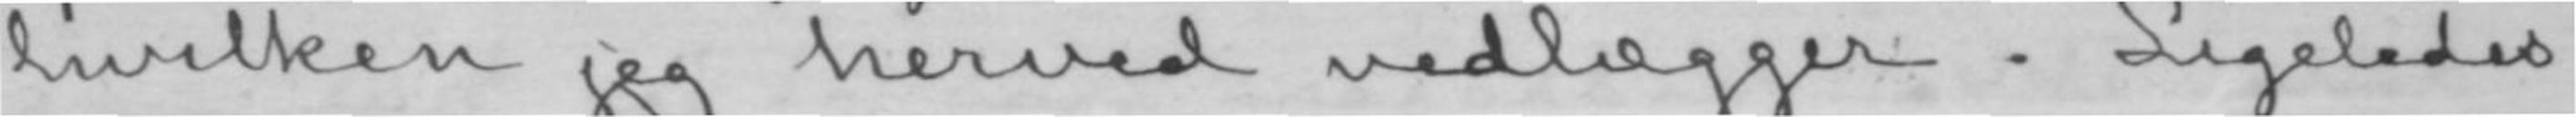hvilken jeg herved vedlægger. Ligeledes
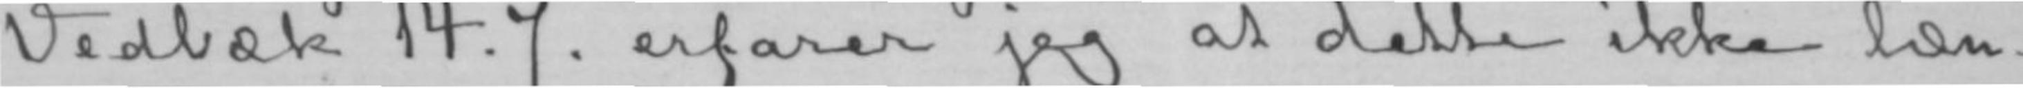Vedbæk 14.7. erfarer jeg at dette ikke læn-
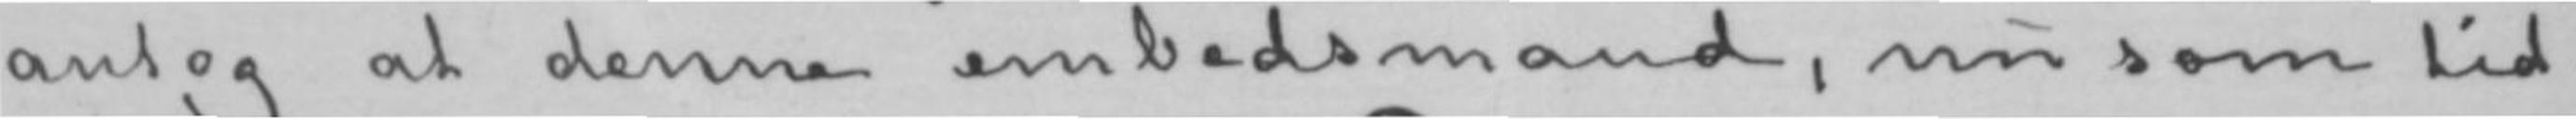antog at denne embedsmand, nu som tid-
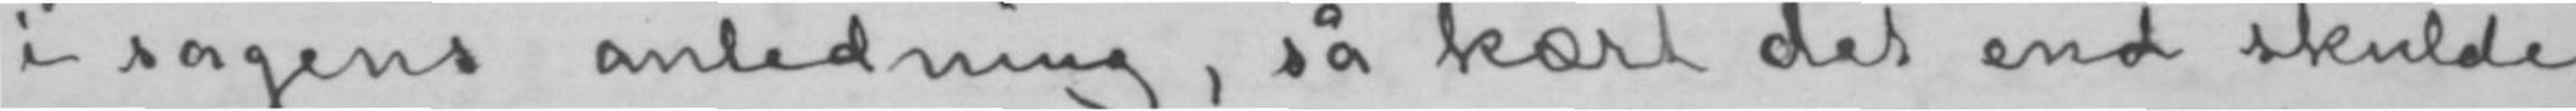i sagens anledning, så kært det end skulde
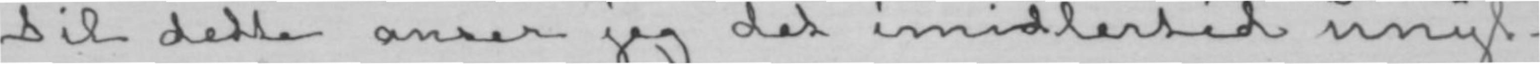Til dette anser jeg det imidlertid unyt-
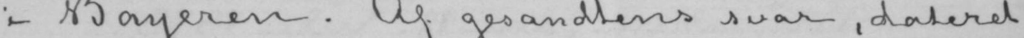i Bayeren. Af gesandtens svar, dateret
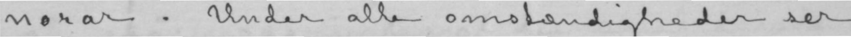norar. Under alle omstændigheder ser
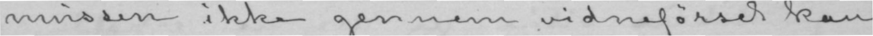mussen ikke gennem vidneførsel kan
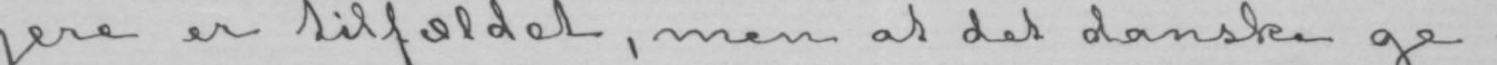gere er tilfældet, men at det danske ge-
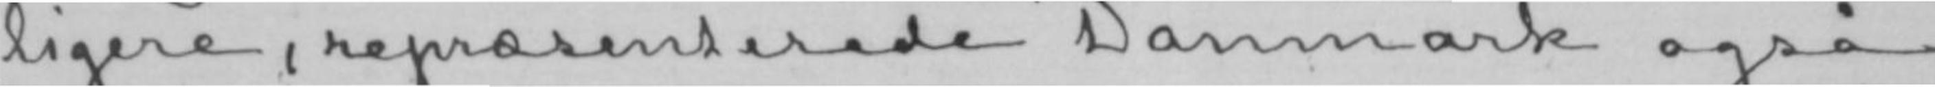ligere, repræsenterede Danmark også
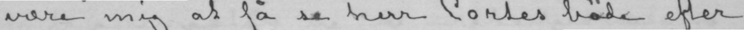være mig at få se herr Cortes bøde efter
